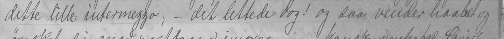dette lille intermezzo; - det lettede dog! og saa vender haabet og
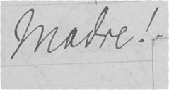madre!
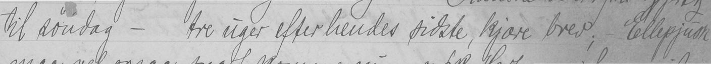til søndag - tre uger efter hendes sidste, kjære brev; Ellepjusk
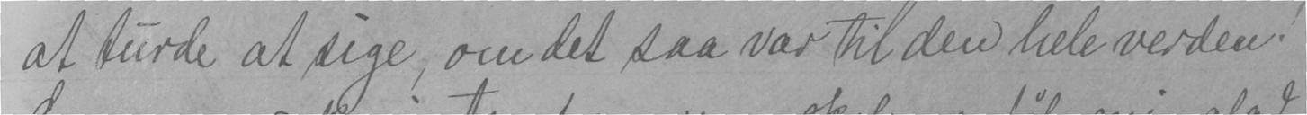at turde at sige, om det saa var til den hele verden!
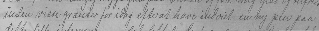inden visse grænser for idag efterat have indviet en ny pen paa
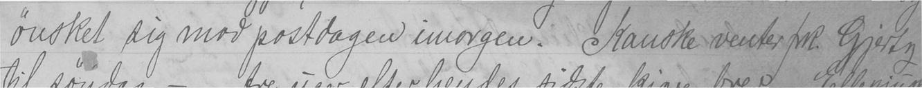ønsket sig mod postdagen imorgen. Kanske venter frk. Gjertz
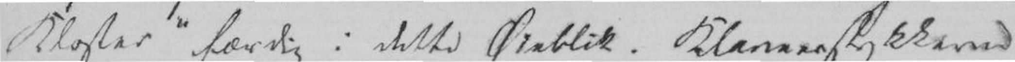Kloster" færdig i dette Øieblik. Klaverstykkerne
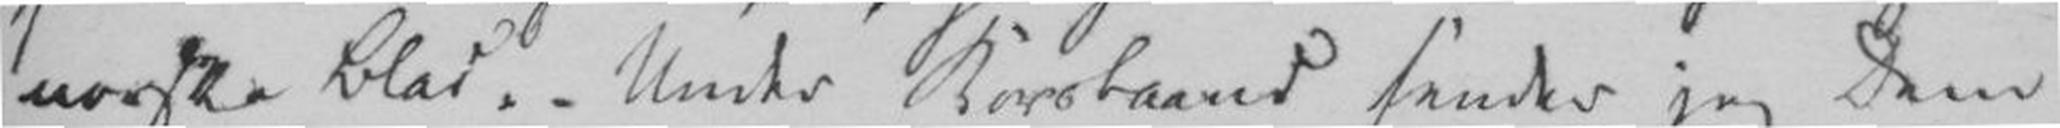norske Blad. Under Korsbaand sender jeg Dem
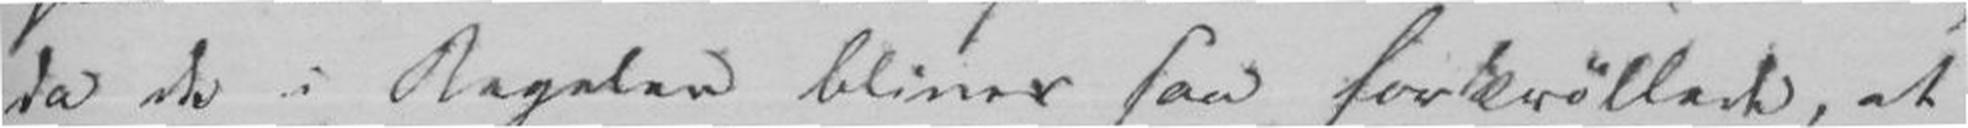da de i Regelen bliver saa forkrøllede, at
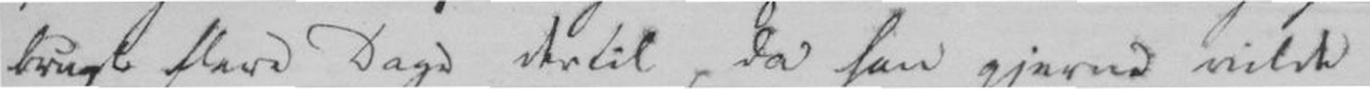brugt flere Dage dertil, da han gjerne vilde
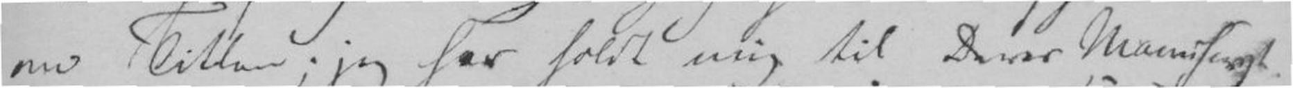om Titlen; jeg har holdt mig til Deres Manuscript.
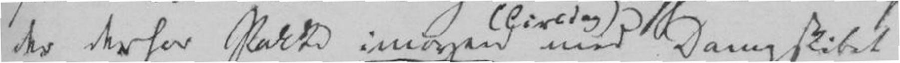der derfor Pakke imogen med Dampskibet
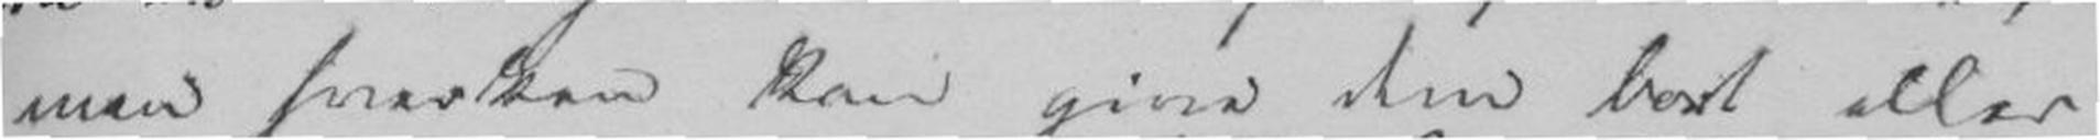man hverken kan give den bort eller
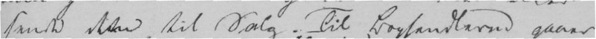sende dem til Salg. Til Boghandleren gaaer
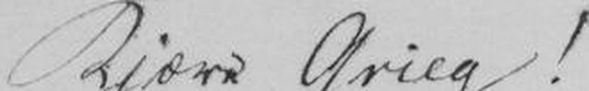Kjære Grieg!
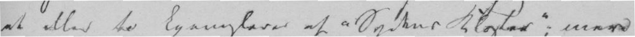et eller to Exemplarer af Sydens Kloster; men
